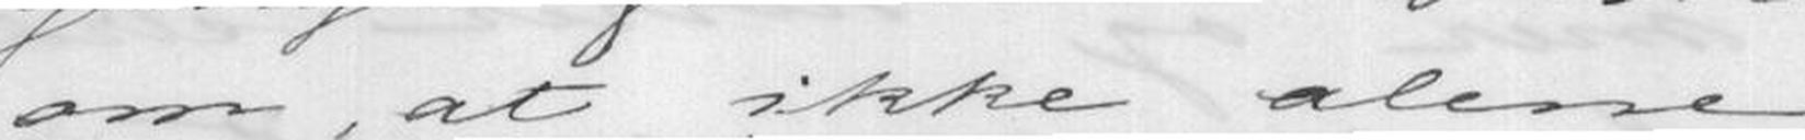om at ikke alene
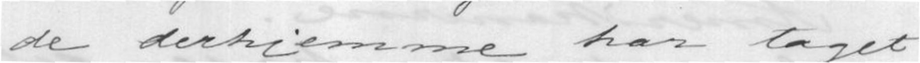de derhjemme har taget
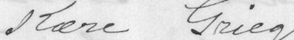Kære Grieg!
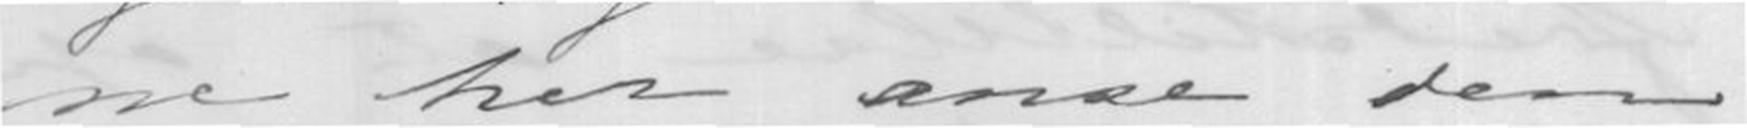ne her anse dem
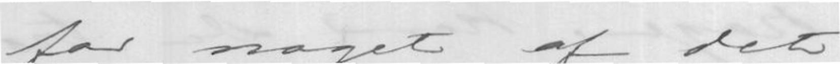for noget af det
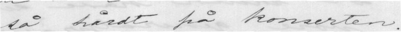så hårdt på konserten.
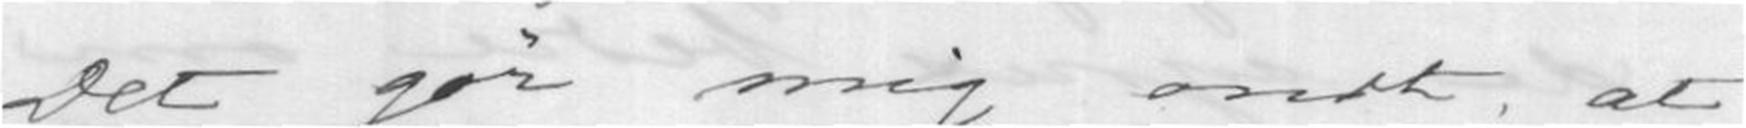Det gør mig ondt, at
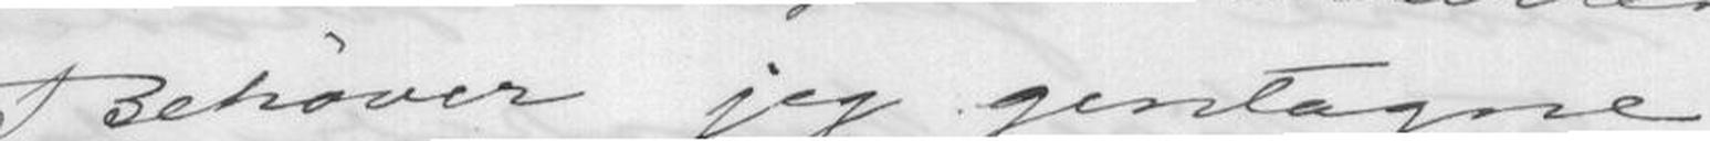Behøver jeg gentagne
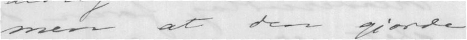men at den gjorde
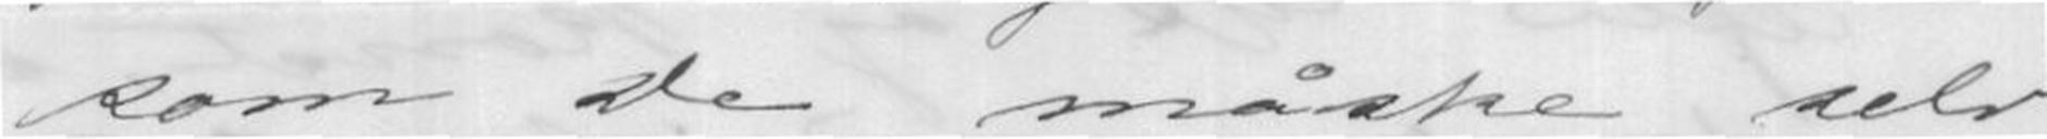som De måske selv
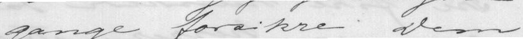gange forsikre Dem
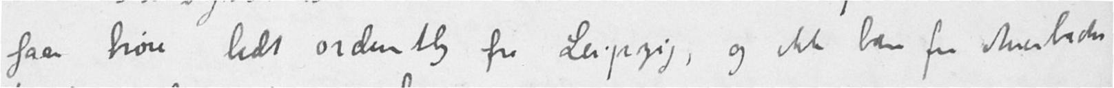faar høre lidt ordentlig fra Leipzig, og ikke bare fra Auerbachs
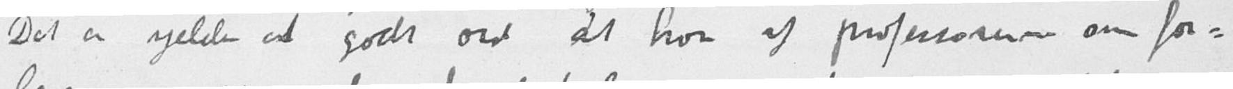Det er sjelden et godt ord at høre af professorerne om for-
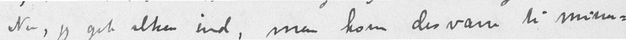Nu, jeg gik altsaa ind, men kom desværre ti minu-
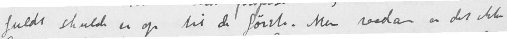fuldt skulde er op til de første. Men saadan er det ikke
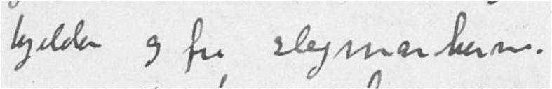kjelder og fra slagmarkerne.
